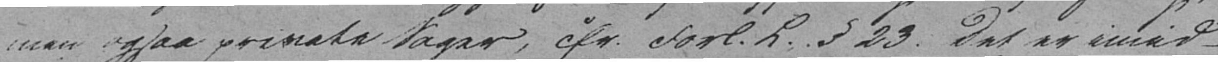men ogsaa private Sager, cfr. Forl. L. § 23. Det er imid-
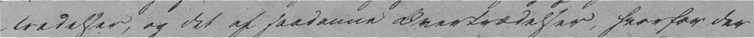trædelser, og det af saadanne Overtrædelser, hvorfor der
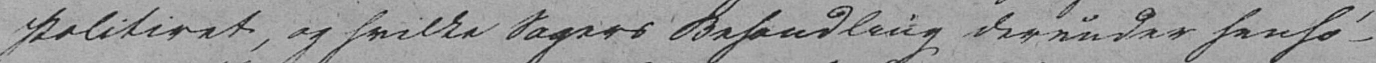Politiret, og hvilke Sagers Behandling derunder henhø-
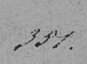351.
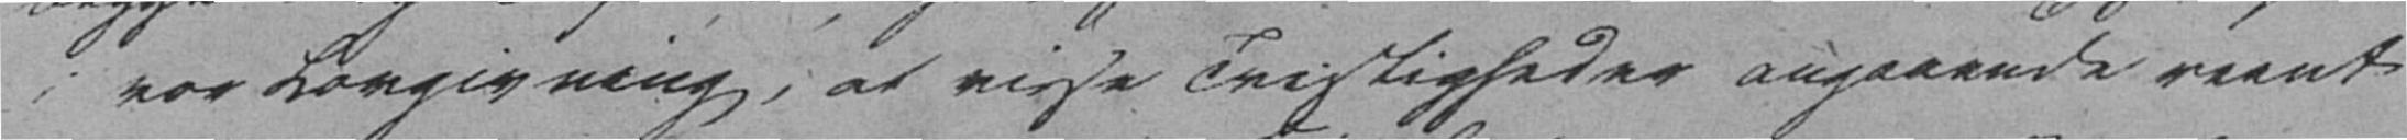i vor Lovgivning, at visse Tvistigheder angaaende reent
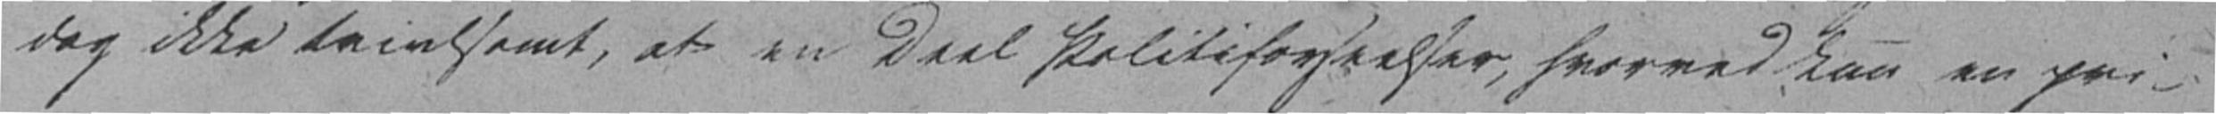dog ikke tvivlsomt, at en Deel Politiforseelser, hvorved kun en pri-
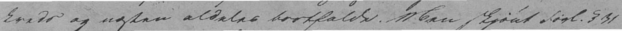kreds og næsten aldeles bortfalde. Men skjønt Forl. § 31
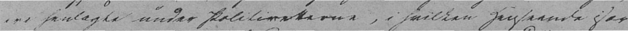ere henlagte under Politiretterne, i hvilken Henseende her
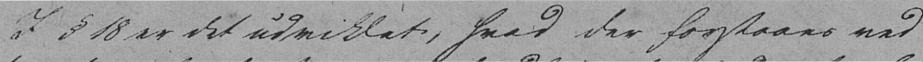I § 18 er det udviklet, hvad der forstaaes ved
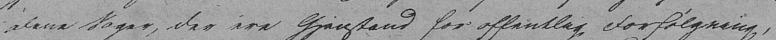alene Sager, der ere Gjenstand for offentlig Forfølgning,
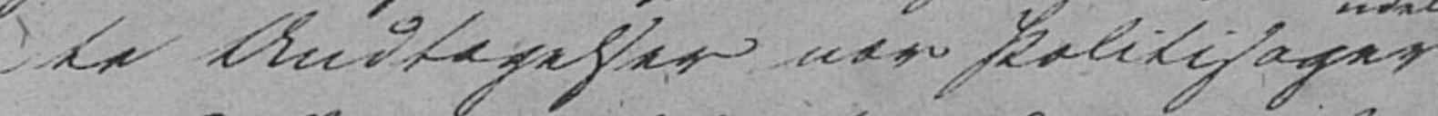te Undtagelser nær Politisager
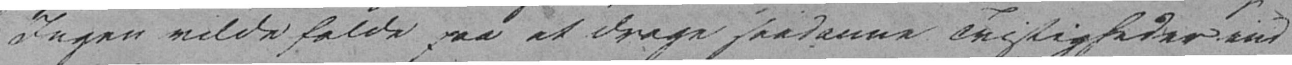Ingen vilde falde paa et drage saadanne Tvistigheder ind
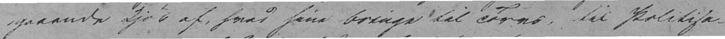gaaende kjøb af, hvad hine bringe til Torvs, til Politisa-
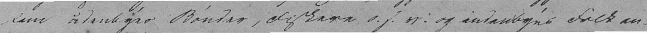lem udenbyes Bønder, Fiskere o.s.v. og indenbyes Folk an-
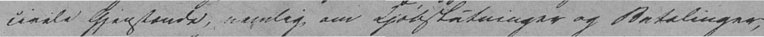civile Gjenstande, nemlig om Kjøbslutninger og Betalinger,
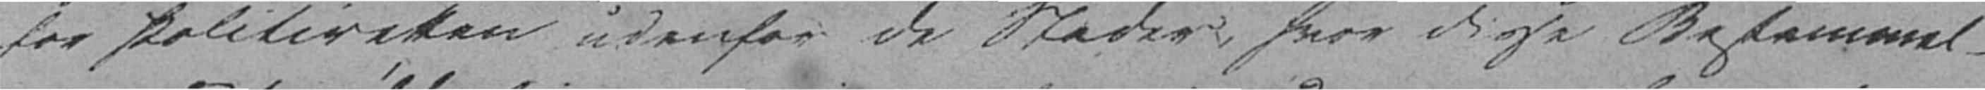for Politiretten udenfor de Steder, hvor disse Bestemmel-
